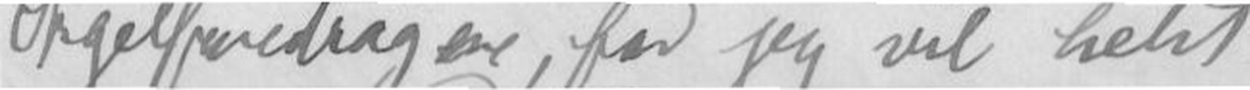Orgelforedragere, for jeg vil helst
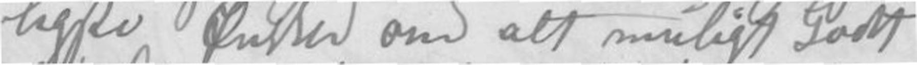ligste Ønsker om alt muligt Godt
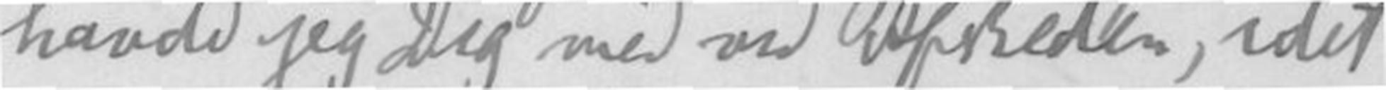havde jeg Dig med ved Afskeden, idet
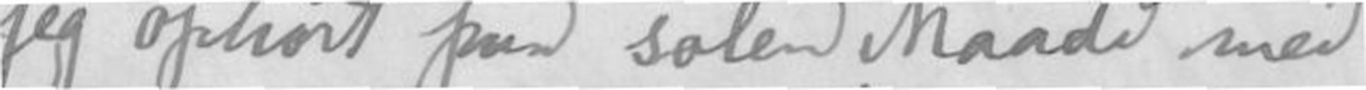jeg ophørt paa solen Maade med
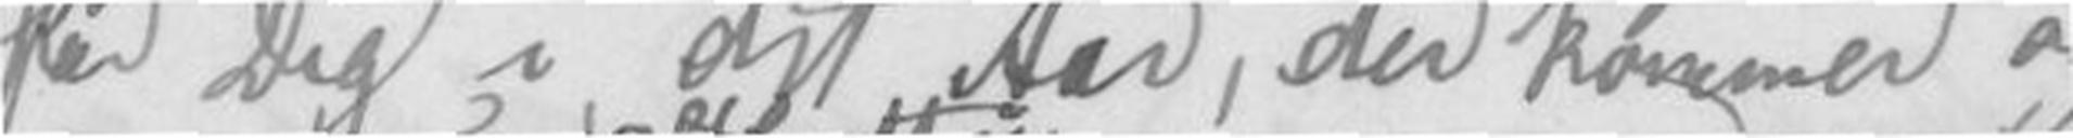for Dig i det Aar, der kommer og
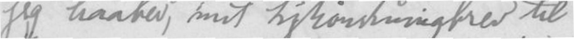jeg haaber, mit Lykønskningsbrev til
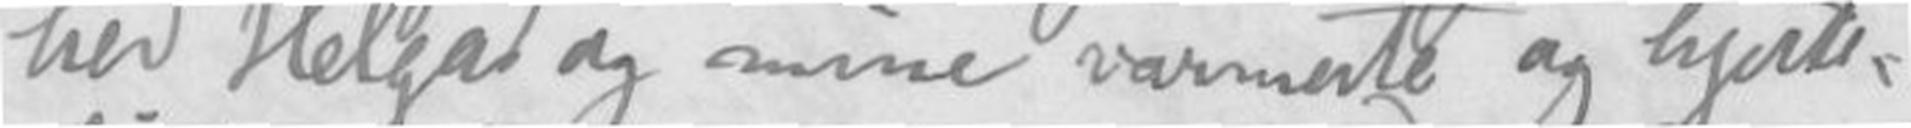her Helgas og mine varmeste og hjerte-
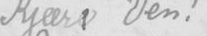Kjære Ven!
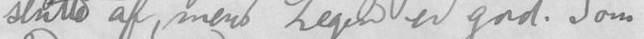slutte af, mens Legen er god. Som
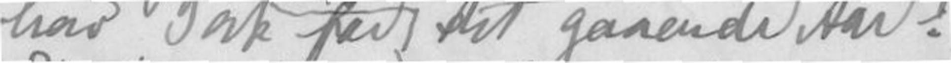hav Tak for alt Godt i det gaaende Aar!
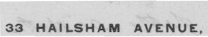33 HAILSHAM AVENUE.
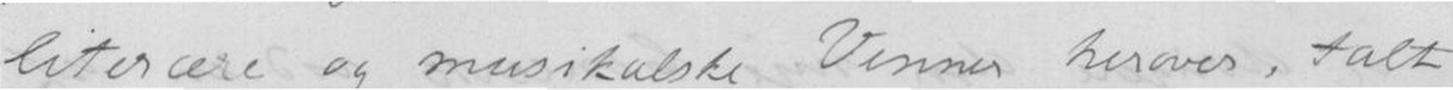literære og musikalske Venner herover, talt
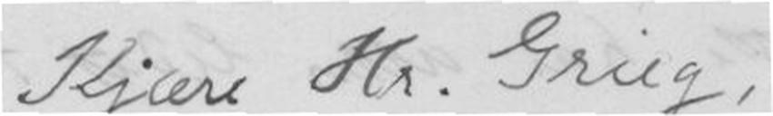Kjære Hr. Grieg.
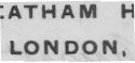LONDON.
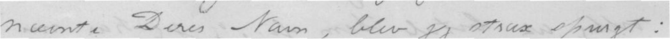nævnte Deres Navn, blev jeg strax spurgt:
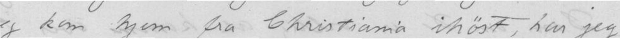jeg kom hjem fra Christiania ihøst, har jeg
